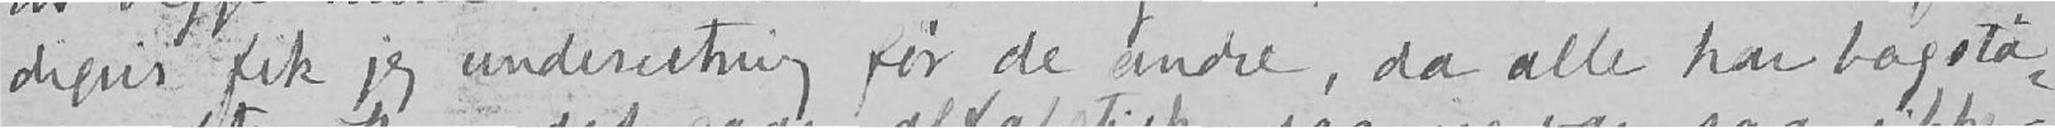digvis fik jeg underretning før de andre, da alle har bogsta-
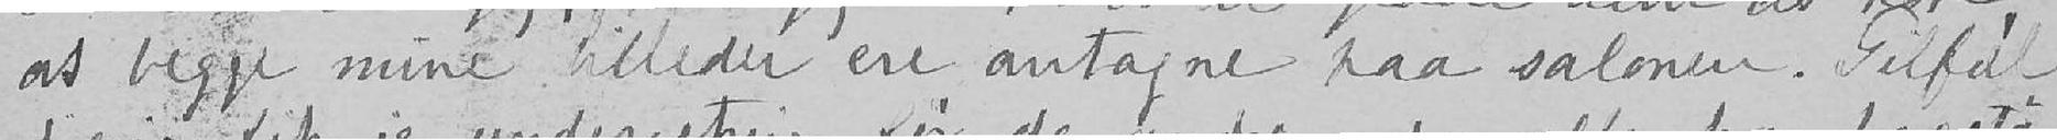at begge mine billeder ere antagne paa salonen. Tilfæl-
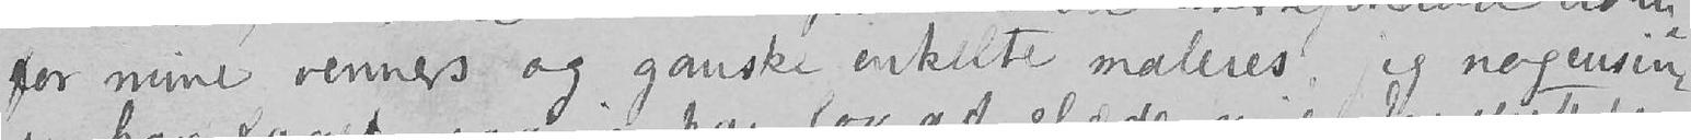for mine venners og ganske enkelte maleres, jeg nogensin-
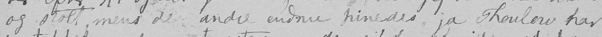og stolt, mens de andre endnu pinedes, ja Thoulow har
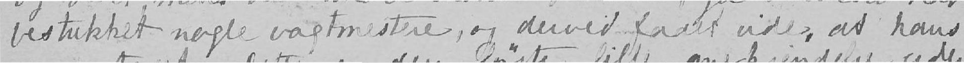bestukket nogle vagtmestere, og derved faaet vide, at hans
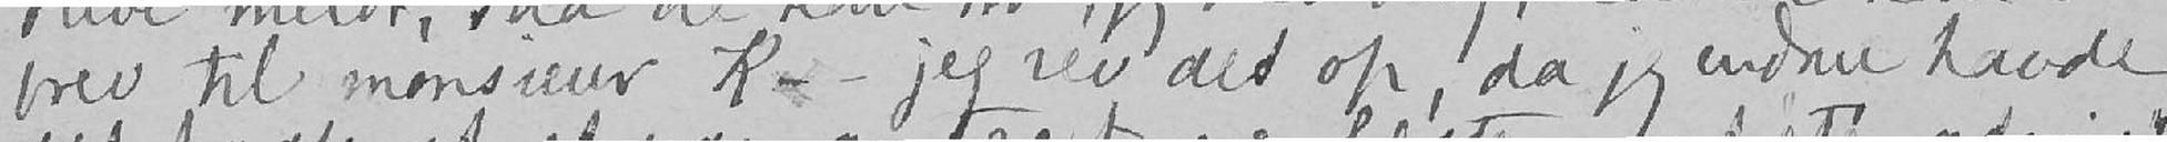brev til monsieur K. – jeg rev det op, da jeg endnu havde
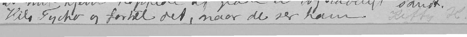Hils Tycho og fortel det, naar de ser ham.
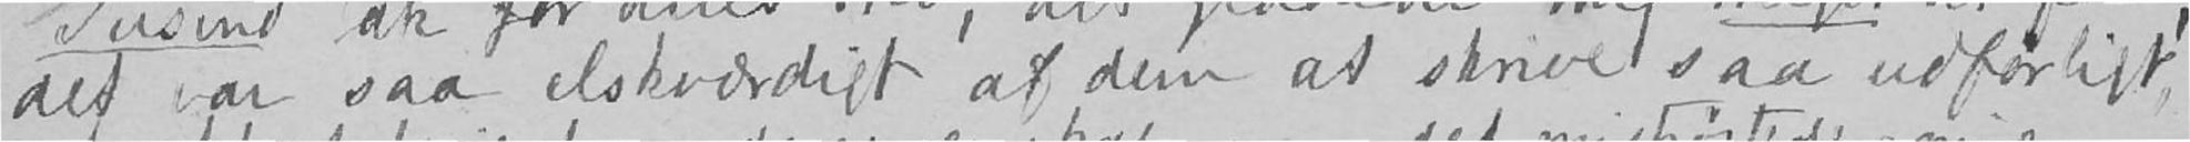det var saa elskværdigt af dem at skrive saa udførligt,
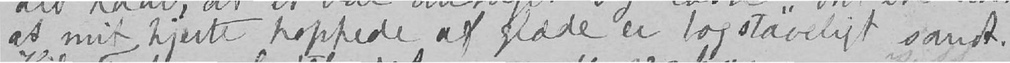at mit hjerte hoppede af glæde er bogstaveligt sandt.
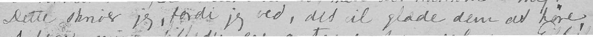Dette skriver jeg, fordi jeg ved, det vil glæde dem at høre,
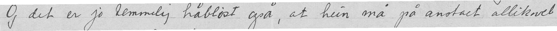Og det er jo temmelig håbløst også, at hun må på anstalt allikevel
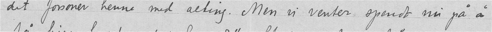det forsoner henne med alting. Men vi venter spendt nu på å
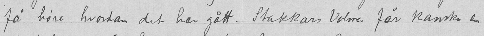få høre hvordan det har gått. Stakkars Volmer får kanske en
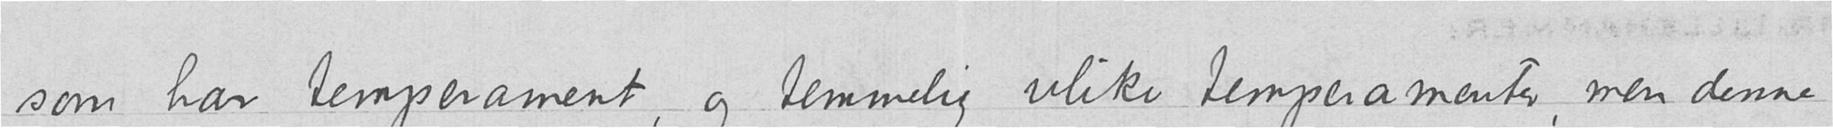som har temperament, og temmelig ulike temperamenter, men denne
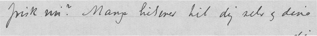frisk nu? Mange hilsener til dig selv og dine
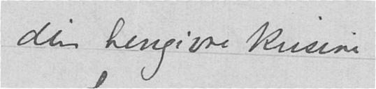din hengivne kusine
